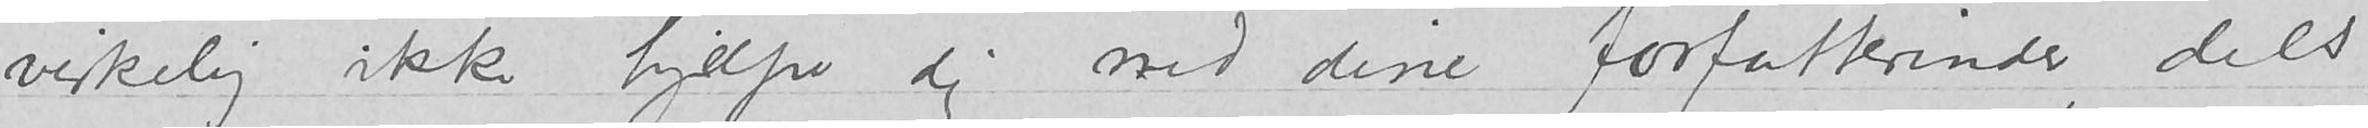virkelig ikke hjelpe dig med dine forfatterinder, dels
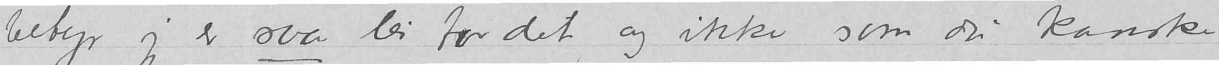betyr jeg er saa lei for det og ikke som du kanske
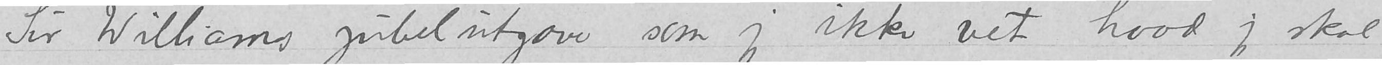Sir Williams jubelutgave som jeg ikke vet hvad jeg skal
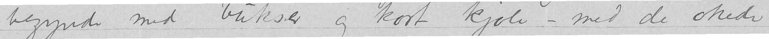begynde med bukser og kort kjole – med de økede
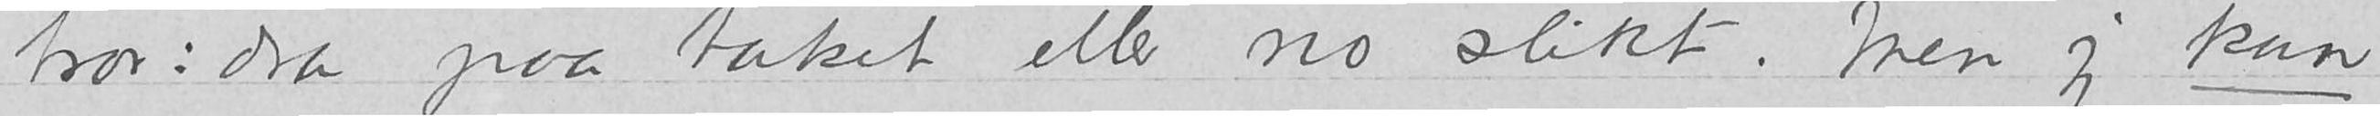tror: dra paa taket eller no slikt. Men jeg kan
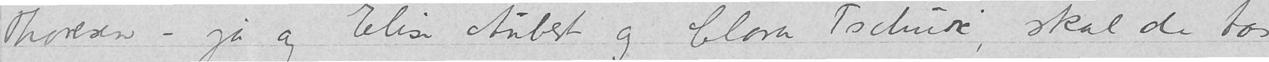Thoresen – jag og Elise Aubert og Clara Tschudi, skal de tas
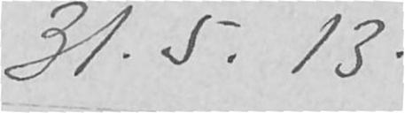31.5.13.
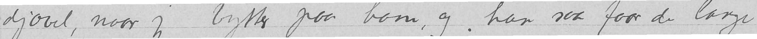djævel, naar jeg bytter paa ham, og han saa faar de lange
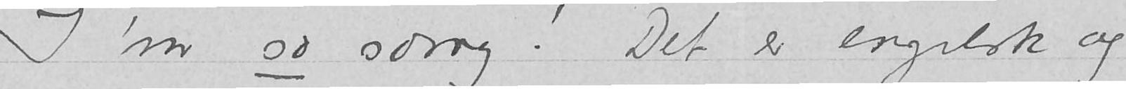I’m so sorry! Det er engelsk og
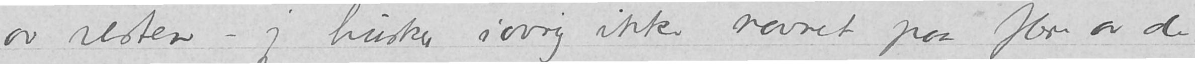av resten – jeg husker iøvrig ikke navnet paa flere av de
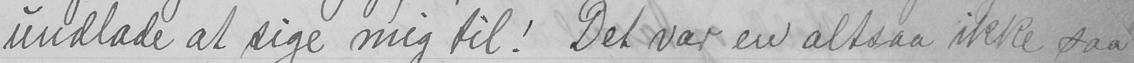undlade at sige mig til! Det var en altsaa ikke saa
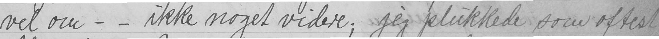vel om - - ikke noget videre; Jeg plukkede som oftest
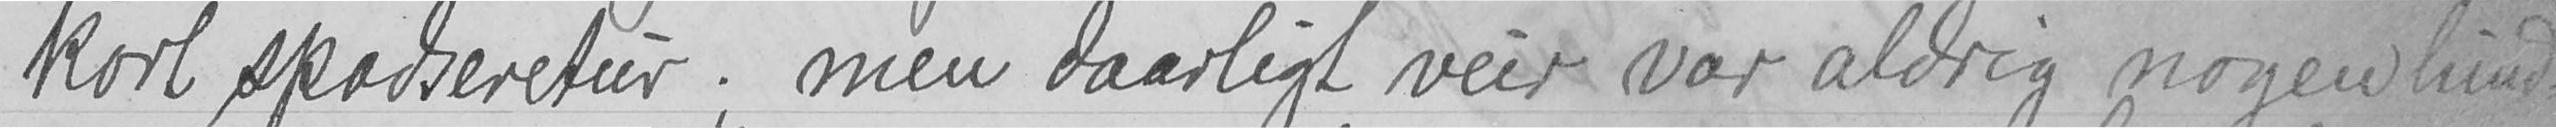kort spadseretur; men daarligt veir var aldrig nonen hind-
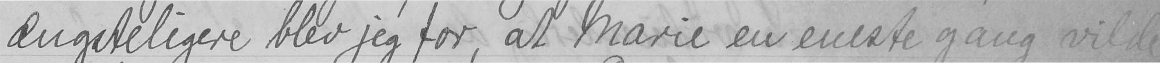ængsteligere blev jeg for, at Marie en eneste gang vilde
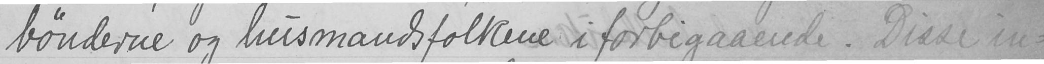bønderne og husmandsfolkene i forbigaaende. Disse in-
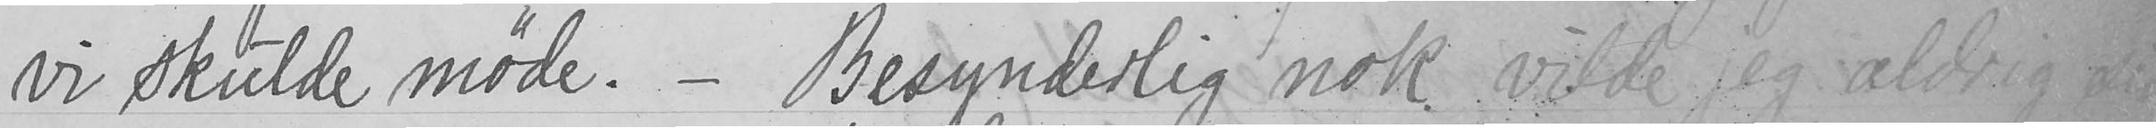vi skulde møde. - Besynderlig nok vilde jeg aldrig si-
